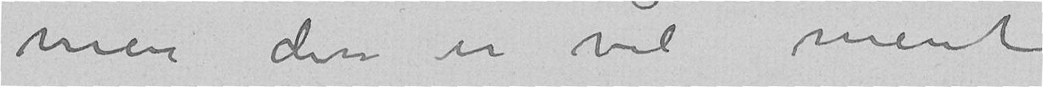men den er vel ment
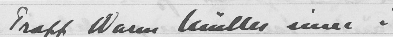Traff Warm Müller inne i
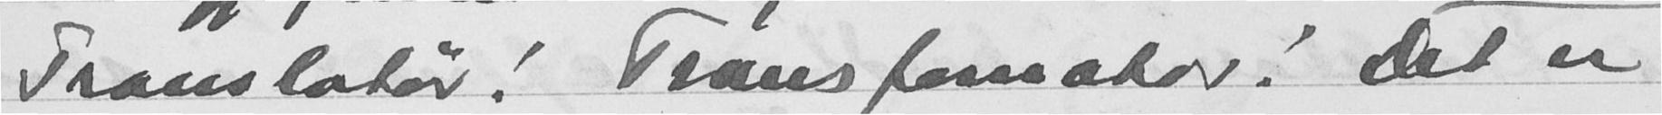Translatør. Transformator! Det er
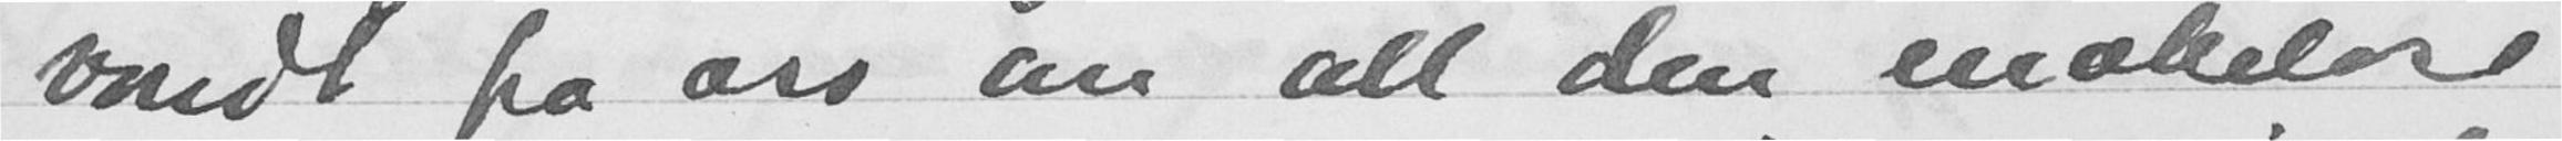vondt fra oss om all den makeløse
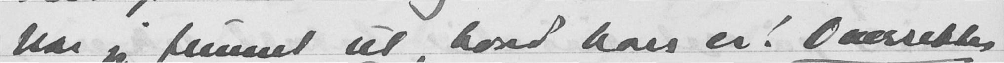har jeg funnet ut, hvad han er! Oversetter
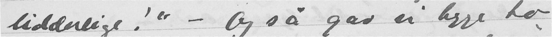lidderlige!" - Og så gav vi begge to
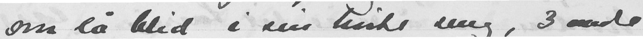som lå blid i sin hvite seng, 3 andre
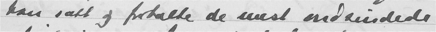han satt og fortalte de mest ondsindede
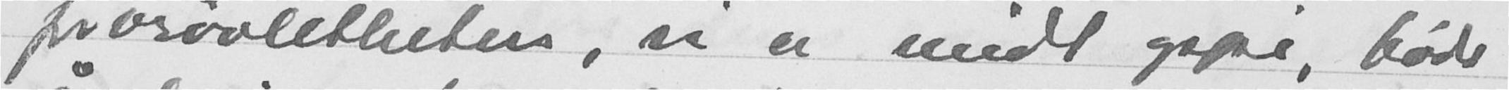frorøvlethetens, vi er midt oppi, både
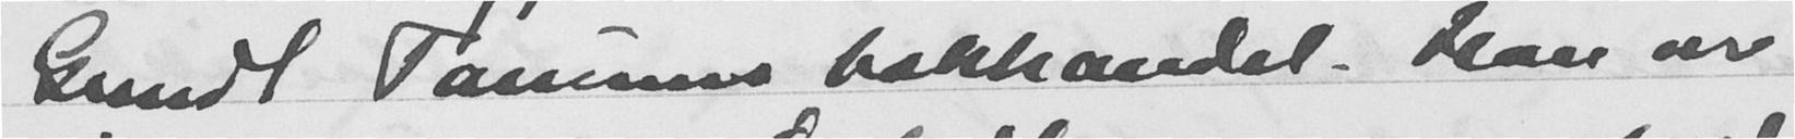Grundt Tanums bokhandel. Han var
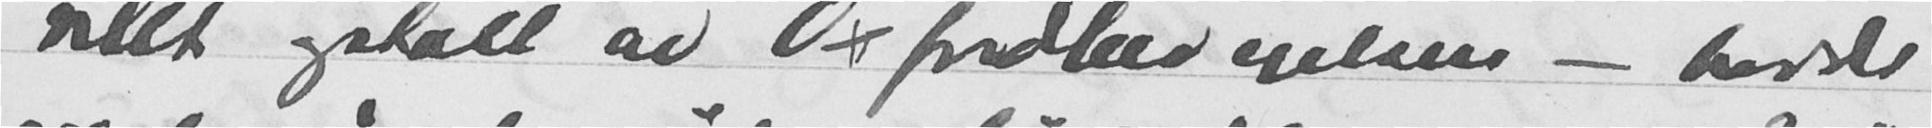villt opptatt av Oxfordbevegelsen - hadde
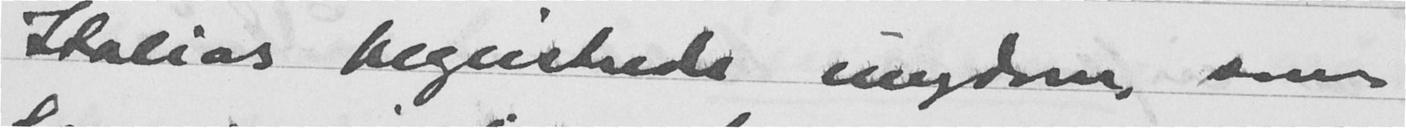Italias begeistrede ungdom, som
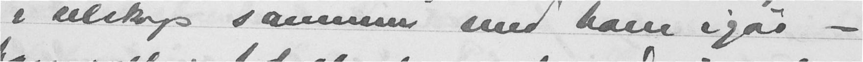i selskap sammen med han igår -
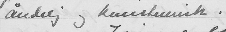åndelig og kunstnerisk.
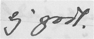sig godt.
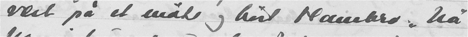vært på et møte og hørt Hambro "nå"
